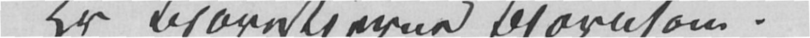Hr Bjørnstjerne Bjørnson!
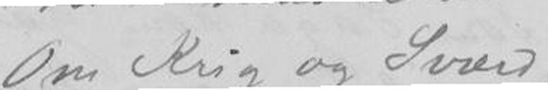Om Krig og Sværd,
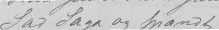Sad Saga og spandt
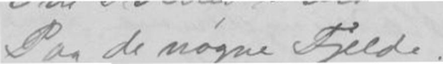Paa de nøgne Fjelde;
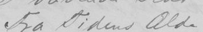Fra Tidens Alde
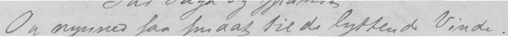Og nynned saa smaat til de lyttende Vinde;
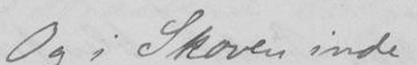Og i Skoven inde
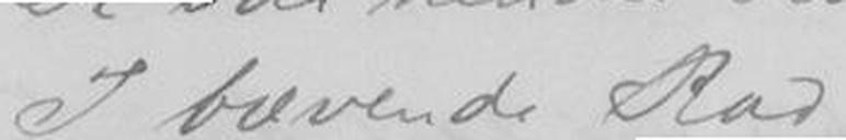I buvende Rad
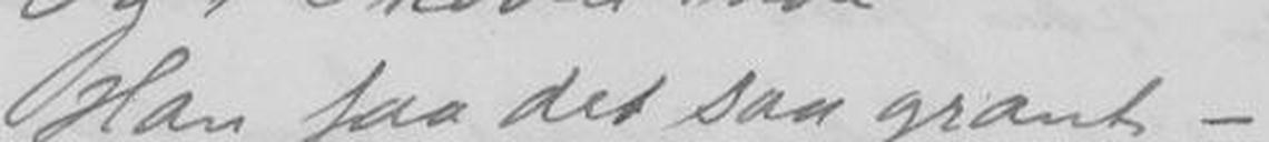-Han saa det som grant -
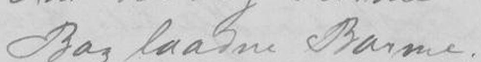Bag laadne Barme;
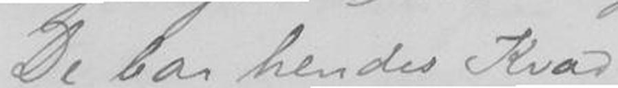De bar hendes Kvad
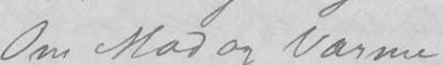Om Mad og Varme
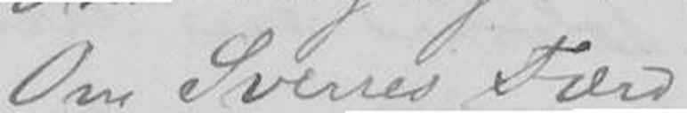Om Sverres Færd
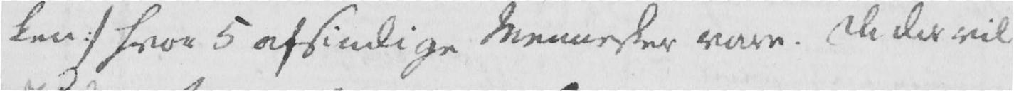ken :/ hvor 5 afsindige Mennesker vare. De der vil
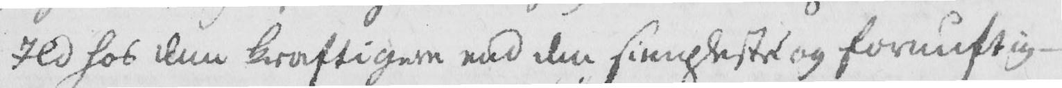Ild hos dem kraftigere end den simpleste og fornuftig-
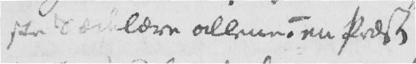ste Sædelære allene. - en Præsts
